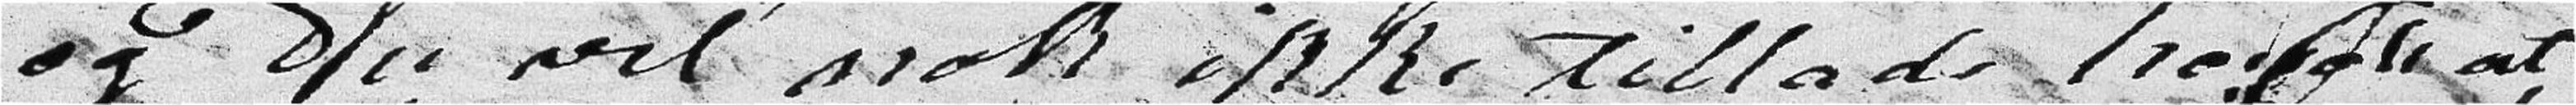og Du vil nok ikke tillade hende at
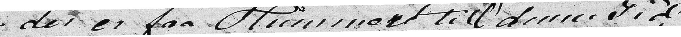der er faa Hummere til denne Tid,
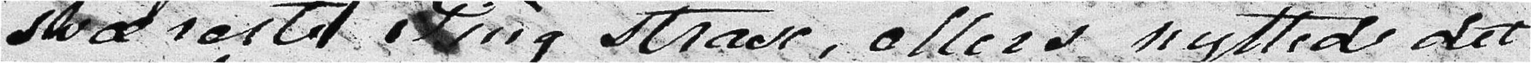sværeste Ting strax, ellers nyttede det
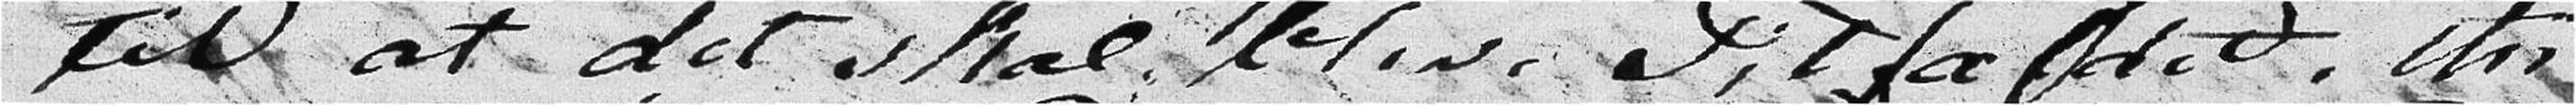til at det skal blive Tilfælde, thi
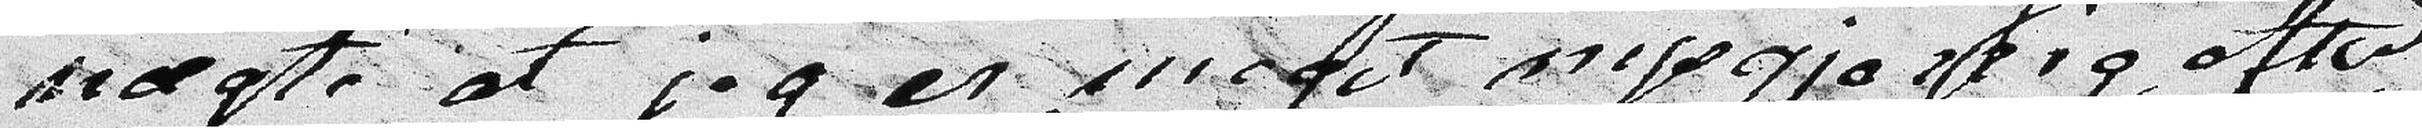nægte at jeg er meget nygerrig efter
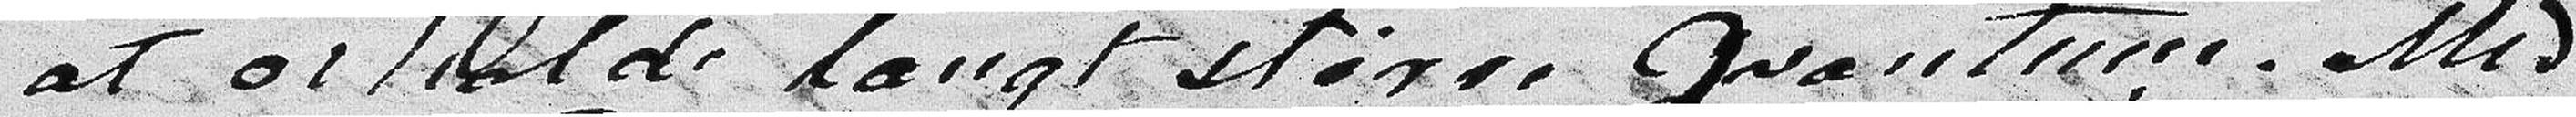at erholde langt større Qvantum. Med
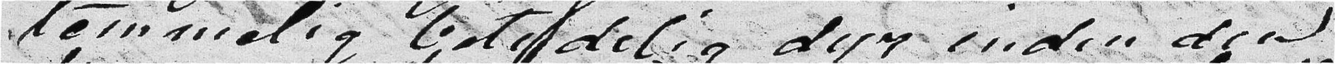temmelig betydelig dyr inden den
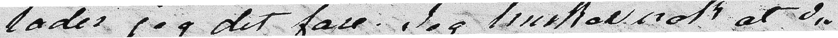lader jeg det fare. Jeg husker nok at Du
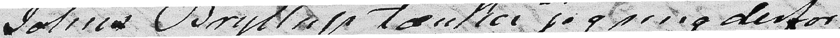John Bryllup tænker jeg mig derfor
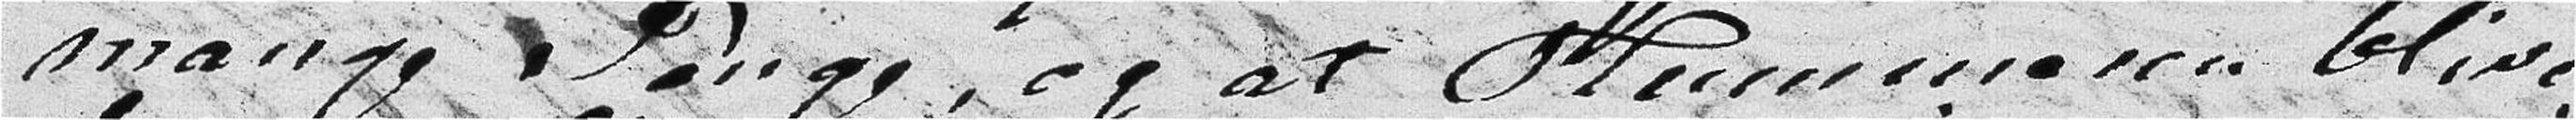mange Penge, og at Hummeren bliver
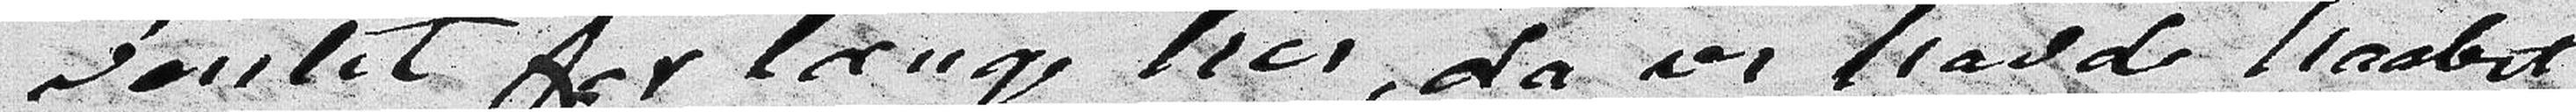ventet for længe her, da vi havde haabet
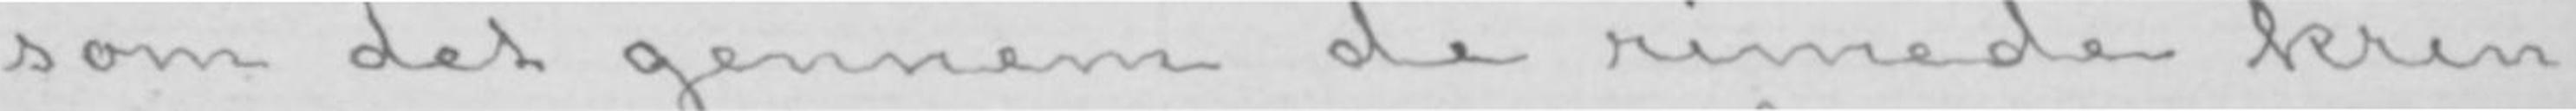som det gennem de rimede krin-
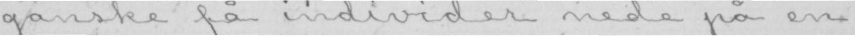ganske få individer nede på en
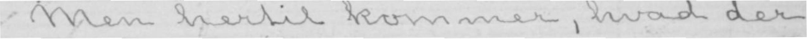Men hertil kommer, hvad der
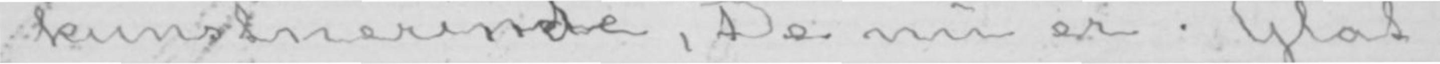kunstnerinde, De nu er. Glat-
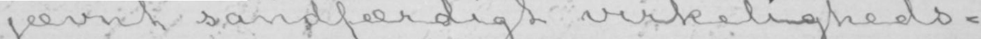jævnt sandfærdigt virkeligheds-
